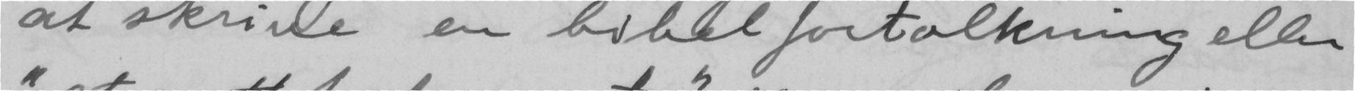at skrive en bibel fortolkning eller
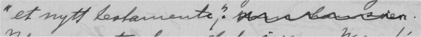"et nytt testamente",.
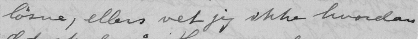løsne, ellers vet jeg ikke hvordan
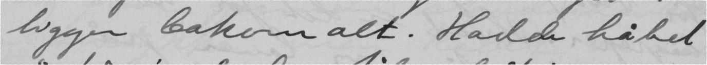ligger bakom alt. Hadde håbet
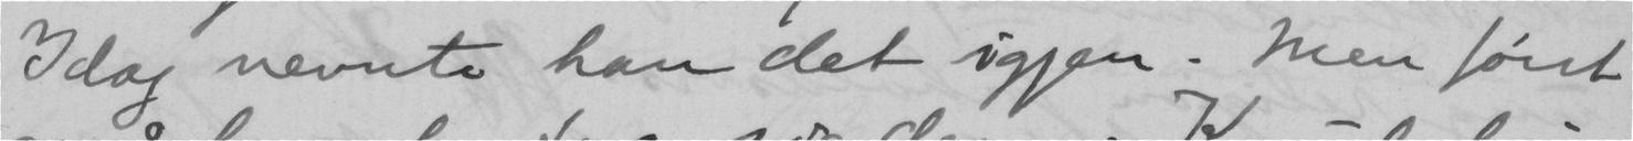Idag nevnte han det igjen. Men først
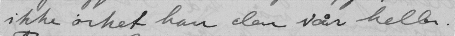ikke orket han den i år heller.
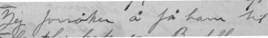Jeg forsøker å få ham til
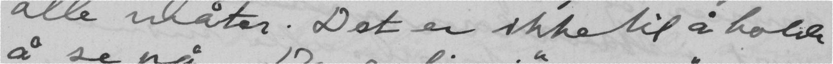alle måter. Det er ikke til å holde
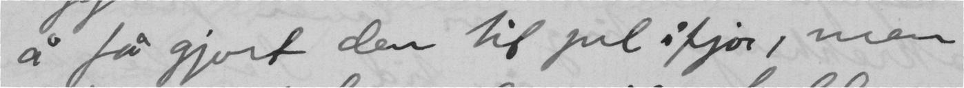å få gjort den til jul i fjor, men
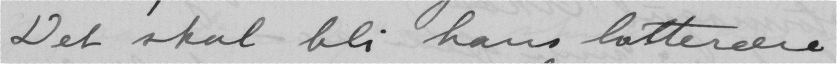Det skal bli hans lattereere
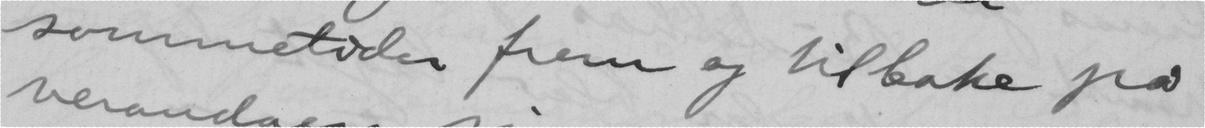sommetider frem og tilbake på
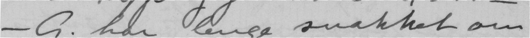- G. har lenge snakket om
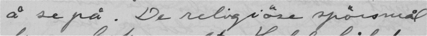å se på. De religiøse spørsmål
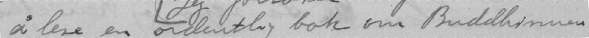å lese en ordentlig bok om Buddhismen
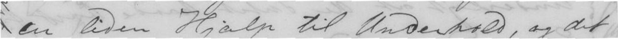en liden Hjælp til Underhold, og det
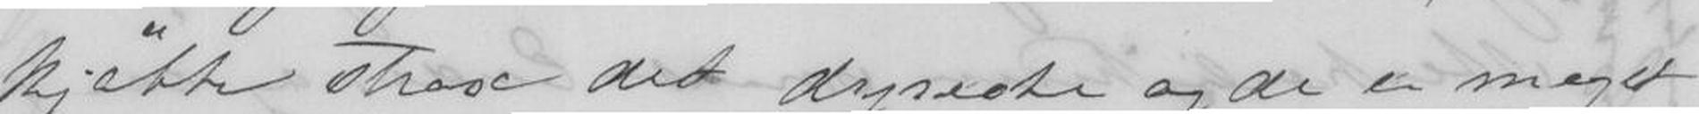kjøbte strax det dyreste og de er meget
